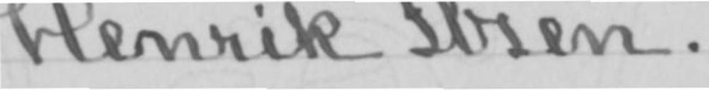Henrik Ibsen.
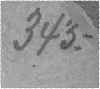343.
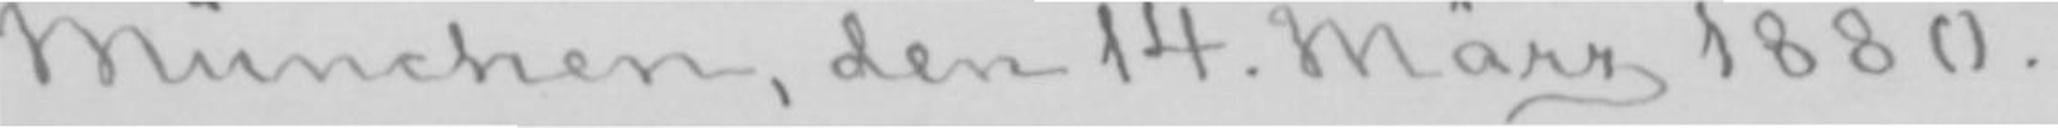München, den 14. März 1880.
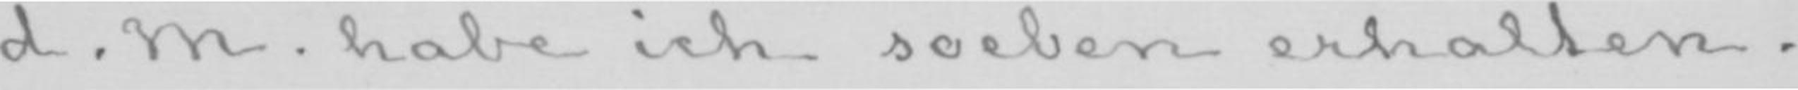d.M. habe ich soeben erhalten.
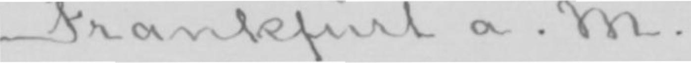Frankfurt a. M.
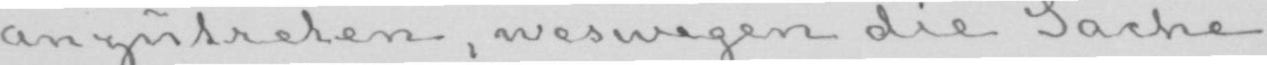anzutreten, weswegen die Sache
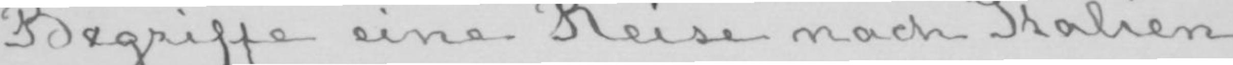Begriffe eine Reise nach Italien
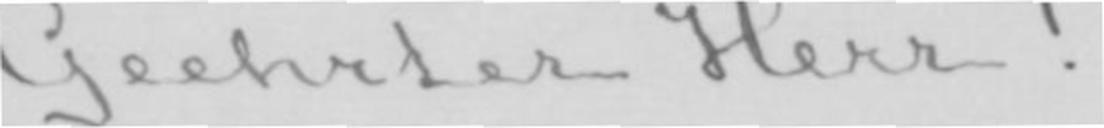Geehrter Herr!
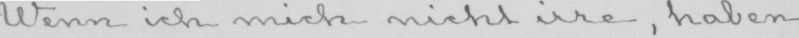Wenn ich mich nicht irre, haben
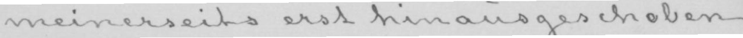meinerseits erst hinausgeschoben
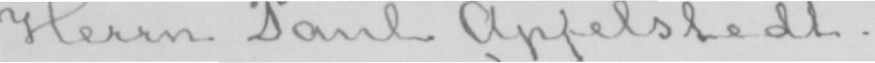Herrn Paul Apfelstedt.
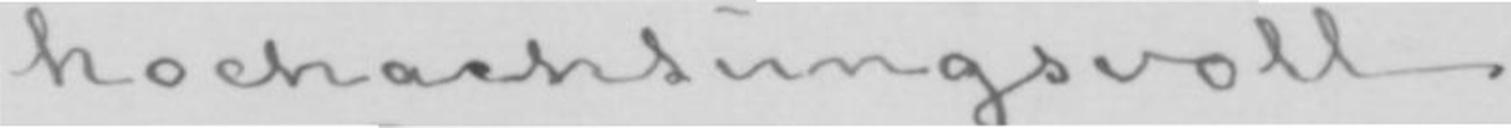hochachtungsvoll
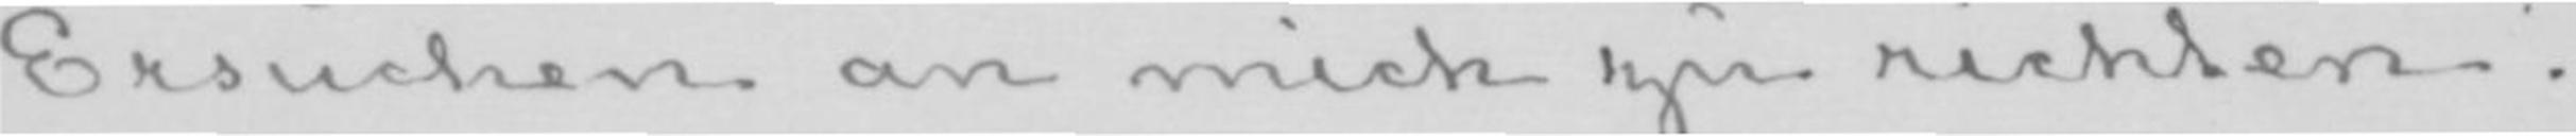Ersuchen an mich zu richten.
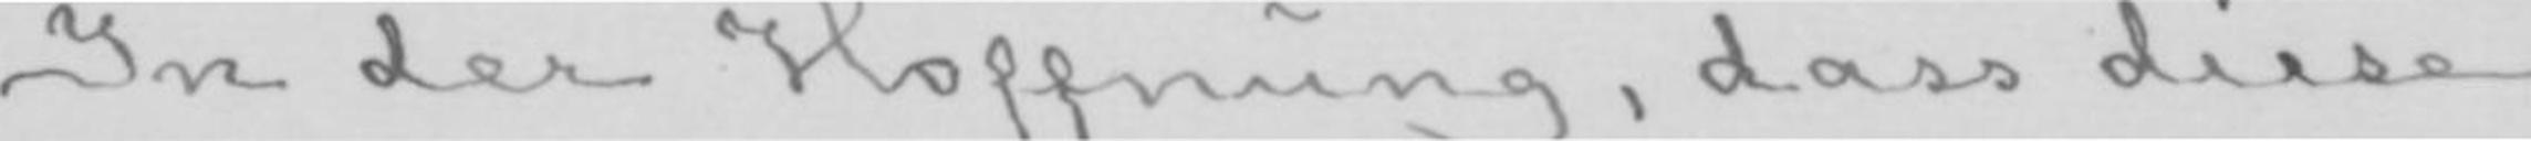In der Hoffnung, dass diese
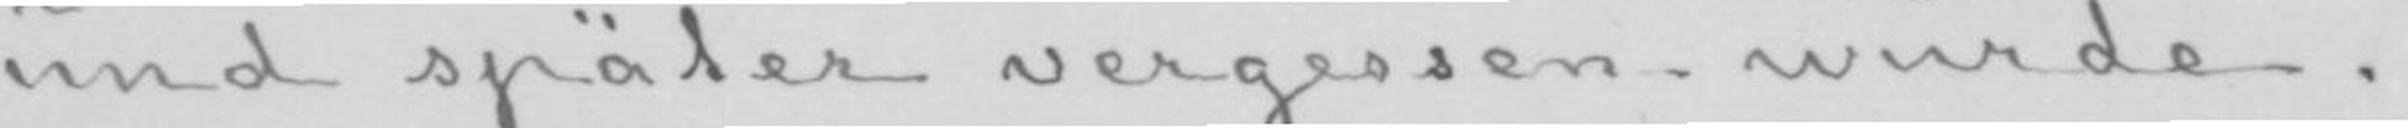und später vergessen wurde.
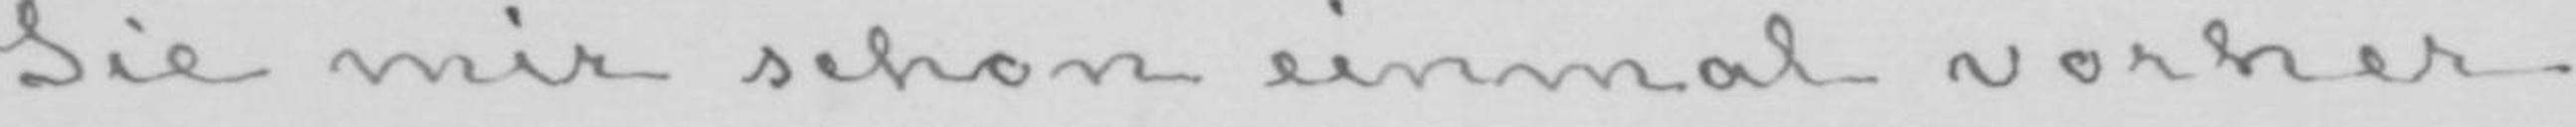Sie mir schon einmal vorher
